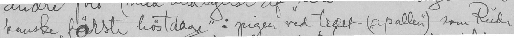kanske "første høstdage" i pigen ved træet ("apallen") som Rudi
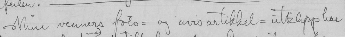Mine venners foto- og avisartikkel- utklipp har
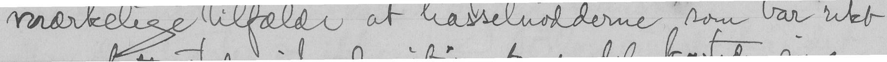mærkelige tilfælde at hasselnødderne som bar rikt
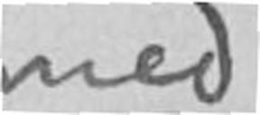med
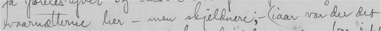vaarnætterne her - men skjeldnere; - (iaar var der det
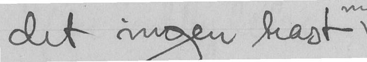det ingen hast
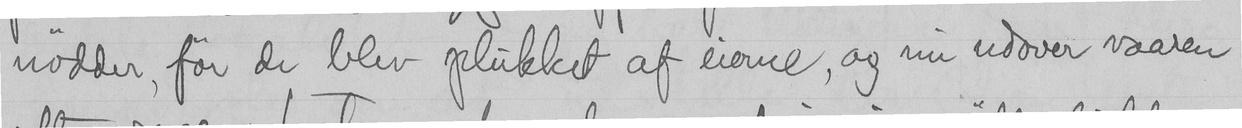nødder, for de blev plukket af eierne, og nu udover vaaren
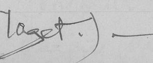taget.) -
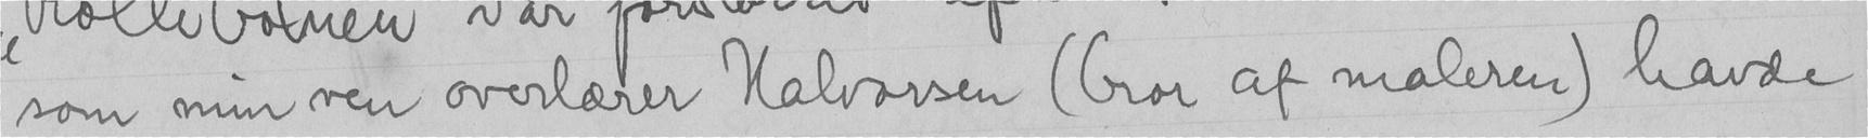som min ven overlærer Halvorsen (bror af maleren) havde
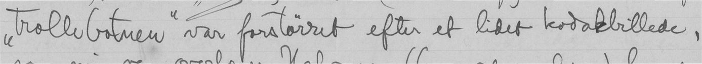"Trollebotnen" var forstørret efter et lidet kodakbillede,
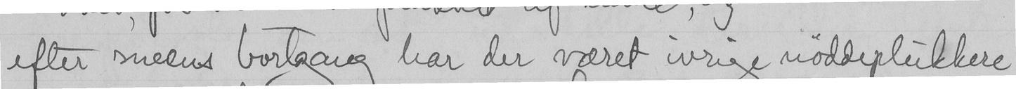efter sneens bortgang har der været ivrige nøddeplukkere
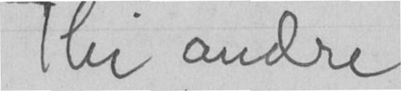thi andre
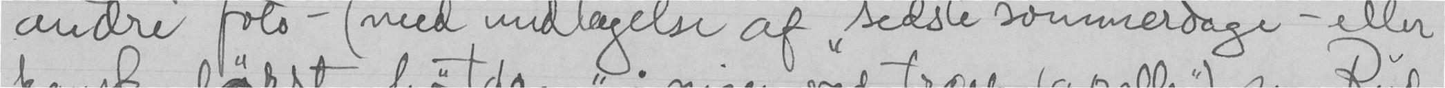andre foto - (med undtagelse af "sidste sommerdage" - eller
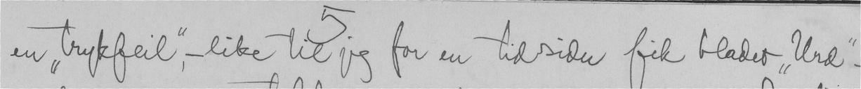en "trykfeil", - like til jeg for en tid siden fik bladet "Urd"-
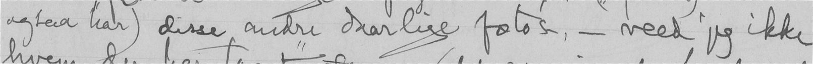ogsaa har) disse andre daarlige fotos, - veed jeg ikke
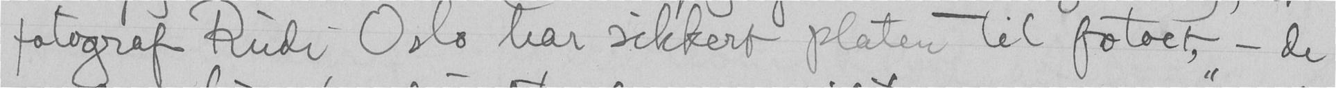fotograf Rudi - Oslo har sikkert platen til fotoet, - de
